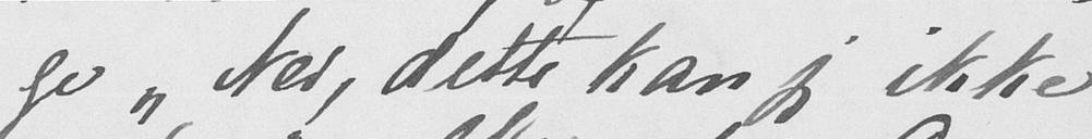ge "Nei, dette kan jeg ikke
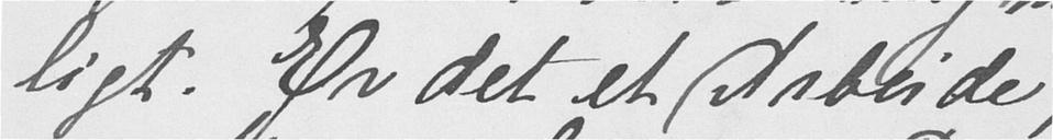ligt. Er det et Arbeide,
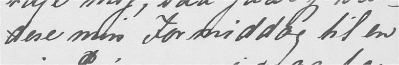dere min Formiddag til en
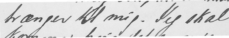trænger til mig. Jeg skal
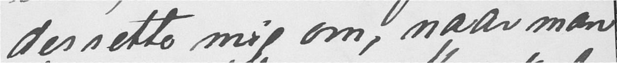derrette mig om, naar man
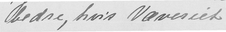bedre, hvis Væveriet
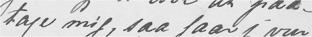tage mig, saa faar jeg vur-
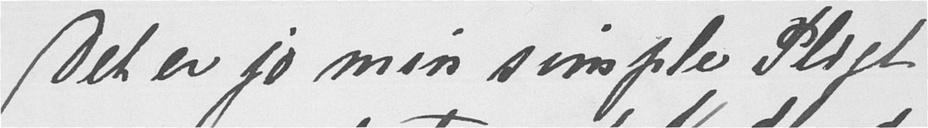Det er jo min simple Pligt
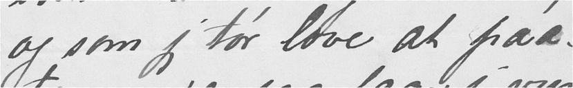og som jeg tør love at paa-
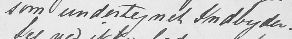som undertegnet Indbyder.
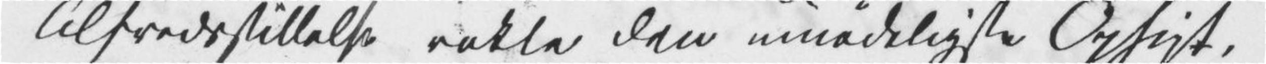Tilfredsstillelse vakte den umådeligste Opsigt,
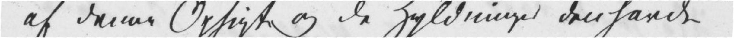af denne Opsigt og de Hyldninger den have
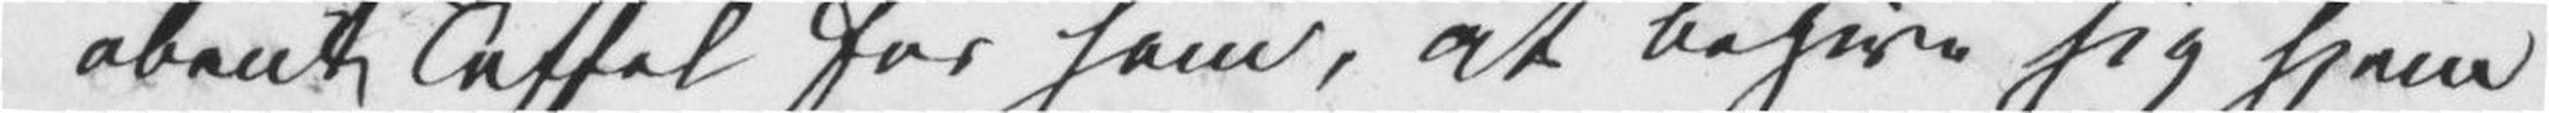åbent Taffel for ham, at begive sig Hjem
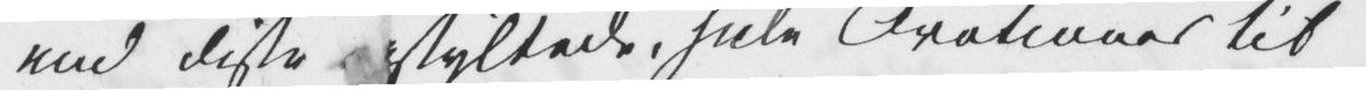end disse styltede, hule Orationer til
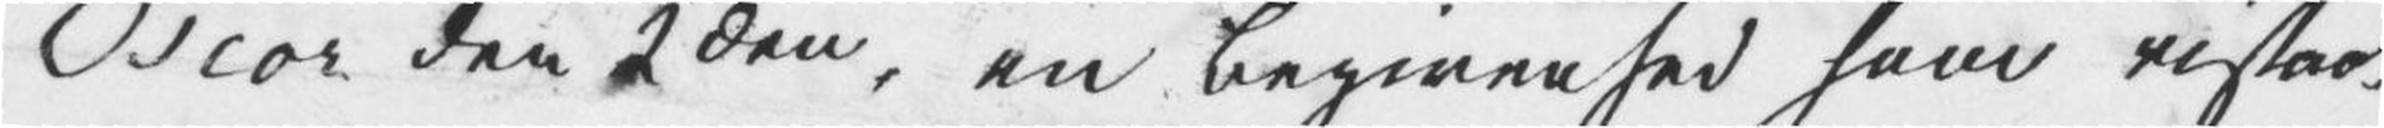Oscar den 2den, en Begivenhed som vistnok
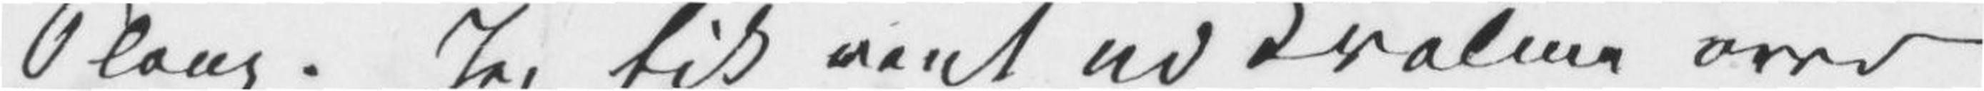Ploug. Jeg fik rent ud Kvalme over
